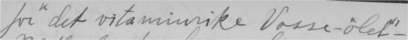for "det vitaminrike Vosse-ölet" —
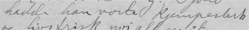hadde han vorte kjempesterk
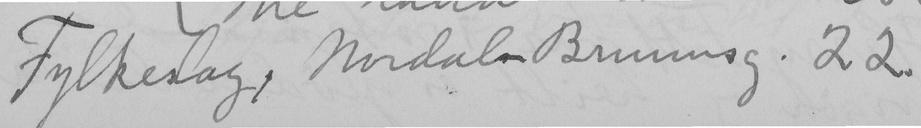Fylkeslag, Nordal Bruunsg. 22.
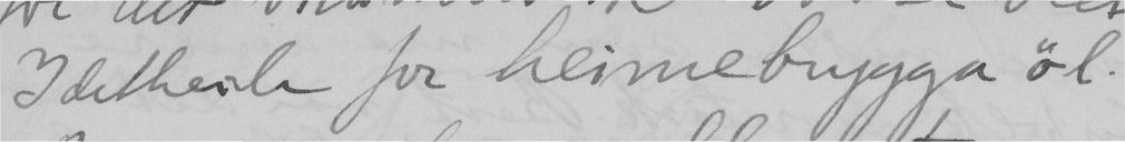I detheila for heimebrygga öl.
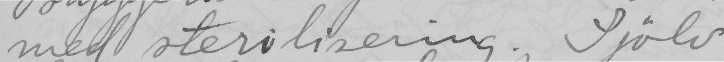med sterilisering. Sjöølv
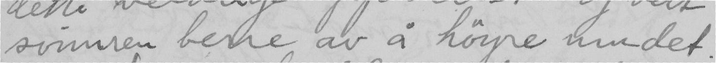svimen berre av å höyre um det.
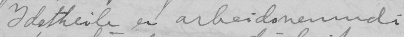Idetheile er arbeidsnemdi
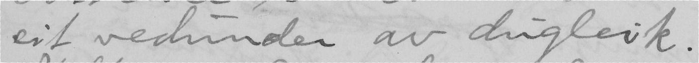eit vedunder av dugleik.
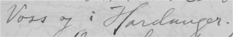Voss og i Hardanger.
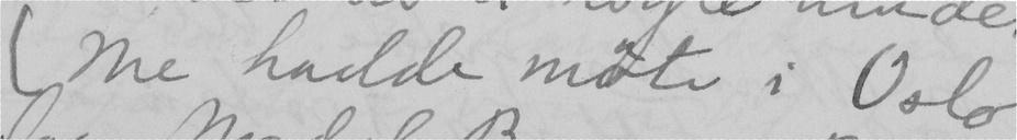Me hadde möte i Oslo
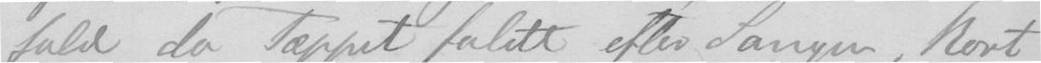fald da Tæppet faldt efter Sangen, kort
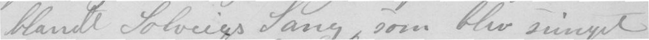blandt Solveigs Sang, som blev sunget
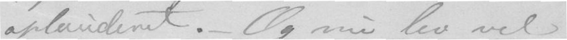aplauderet. - Og nu lev vel
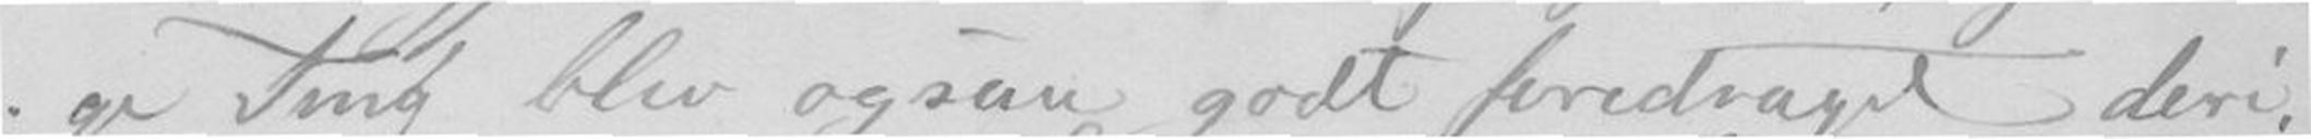ge Ting blev ogsaa godt foredraget deri-
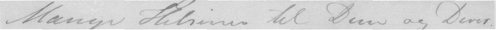Mange Hilsener til Dem og Deres
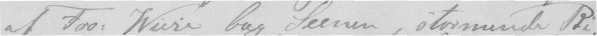af Fro: Wiese bag Scenen, stormende Bi-
